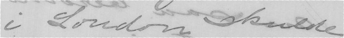i London skulde
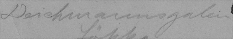Deicmansgaten
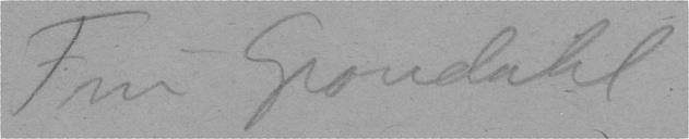Fru Grøndahl
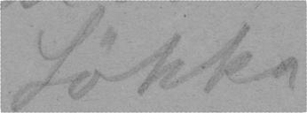Løkka
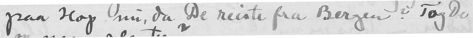paa Hop nu, da De reiste fra Bergen? Tog De
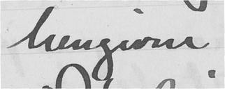hengivne
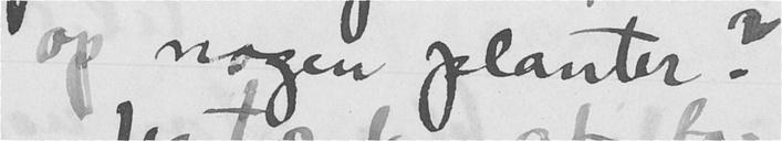op nogen planter?
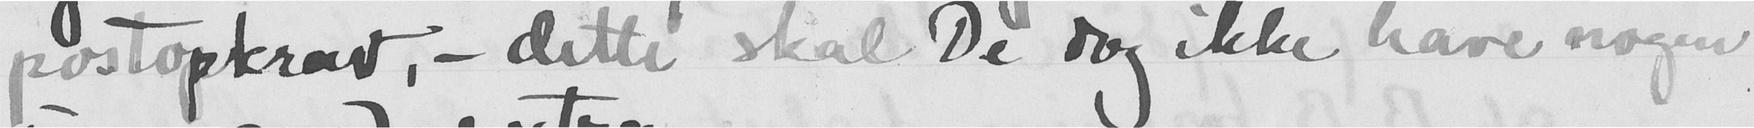postopkrav, - dette skal De dog ikke have nogen
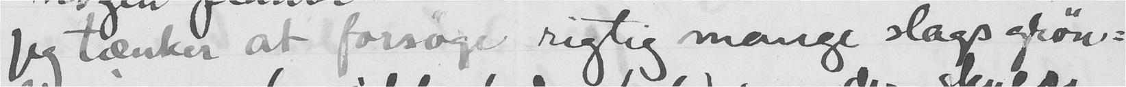Jeg tænker at forsøge rigtig mange slags grøn-
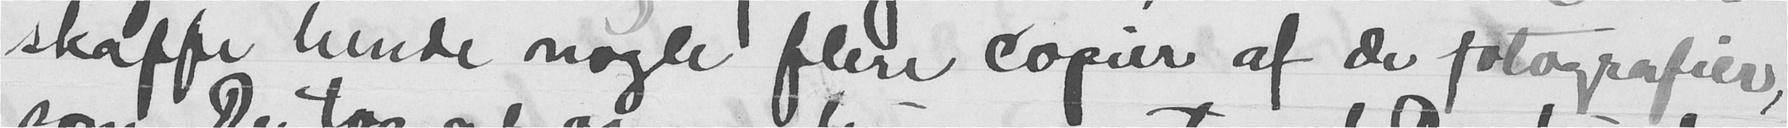skaffe hende nogle flere copier af de fotografier,
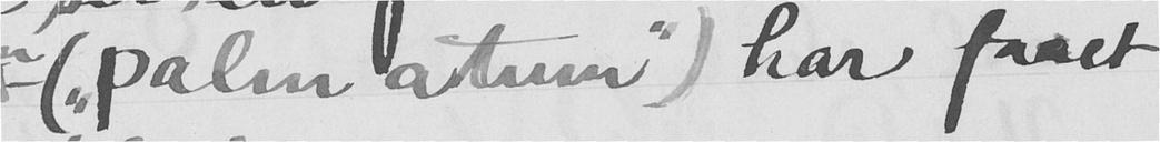("palm atum") har faaet
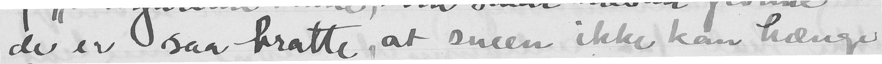de er saa bratte, at sneen ikke kan hænge
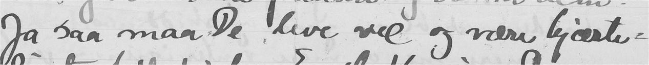Ja saa maa De leve vel og være hjærte-
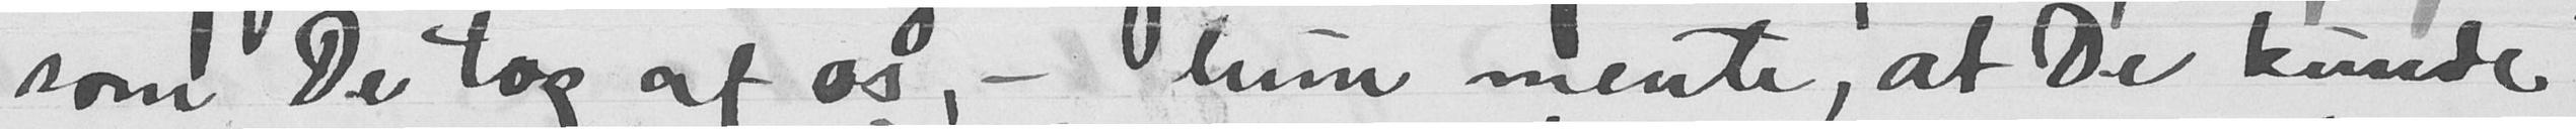som De tog av os, - hun mente, at De kunde
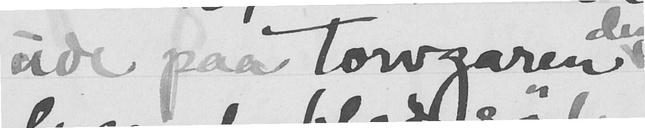ude paa torvgaren
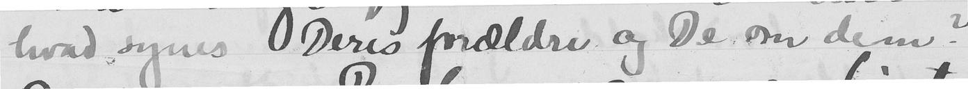hvad synes Deres forældre og De om dem?
